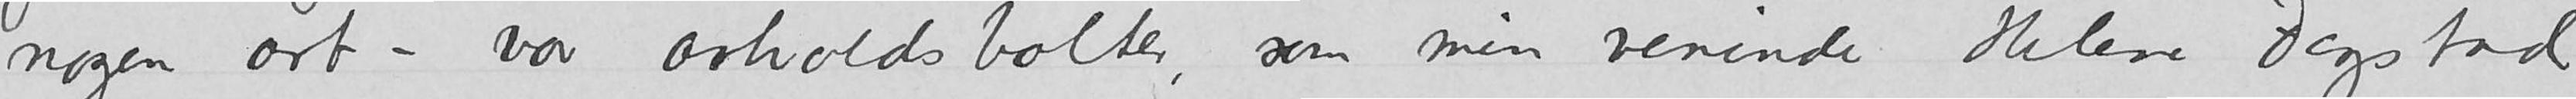nogen art – var avholdsbolter, som min veninde Helene Jegstad
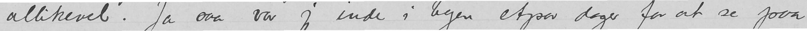allikevel. Ja saa var jeg inde i byen etpar dager for at se paa
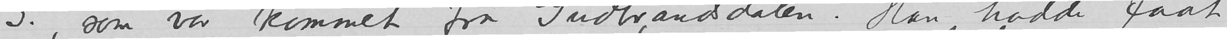S. som var kommet fra Gudbransdalen. Han hadde faat
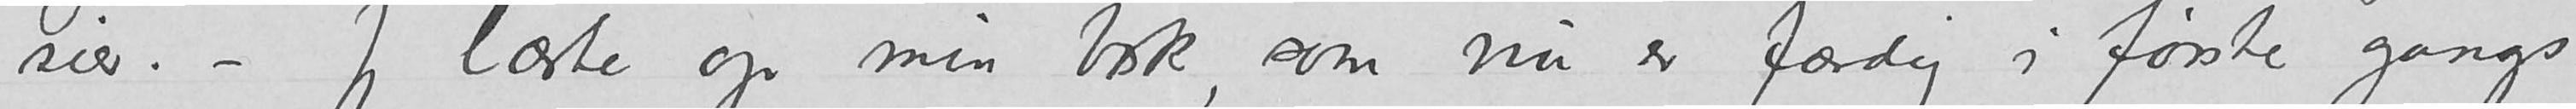sier. – Jeg læste op min bok, som nu er færdig i første gangs
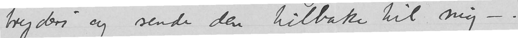bryderi og sende den tilbake til mig -.
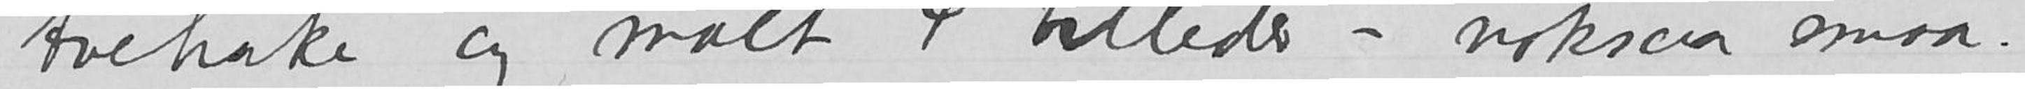tvehake og malt 4 billeder – noksaa smaa
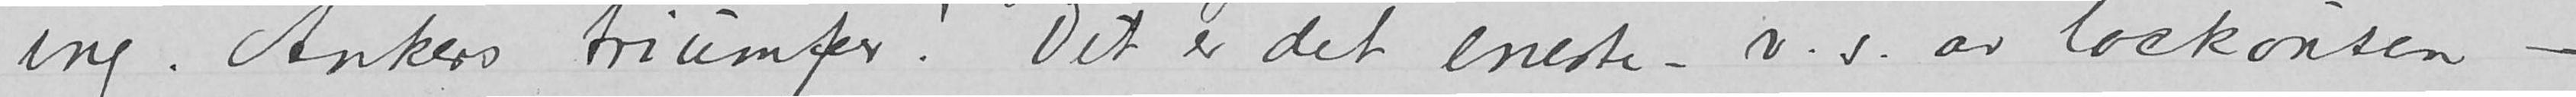ing. Ankers triumfer! Det er det eneste – v. s. av lockouten  -
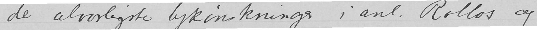de alvorligste lykønskninger i anl. Rollos og
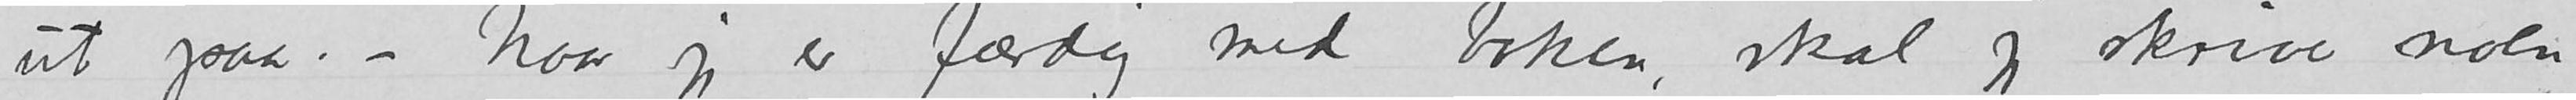ut paa. – Naar jeg er færdig med boken, skal jeg skrive noen
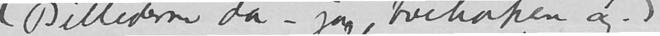(Billederne da - ja tvehaken og.)
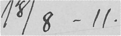18/8 -11.
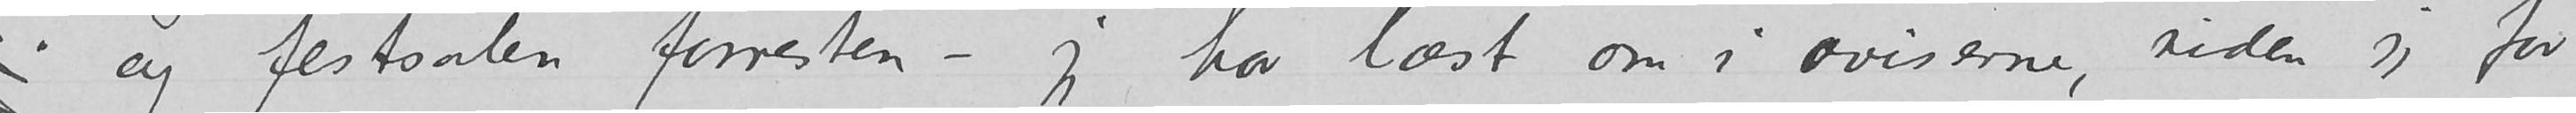og festsalen forresten – jeg har læst om i aviserne, siden jeg for
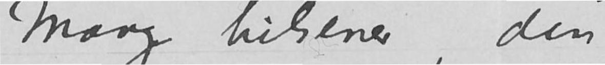Mange hilsener, din
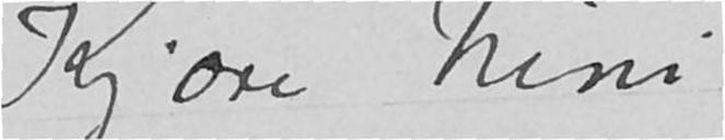Kjære Nini,
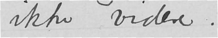ikke videre.
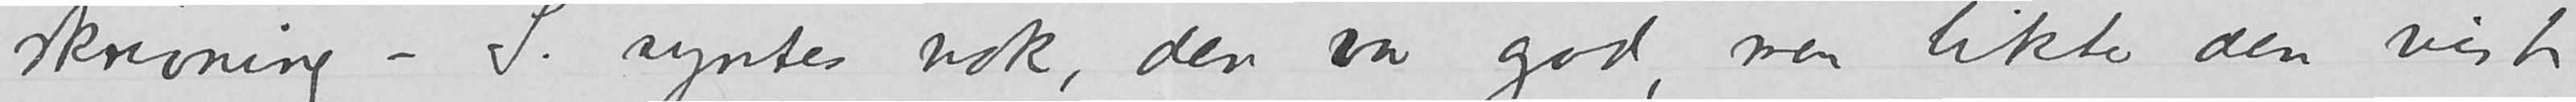skrivning – S. syntes nok, den var god, men likte den vist
